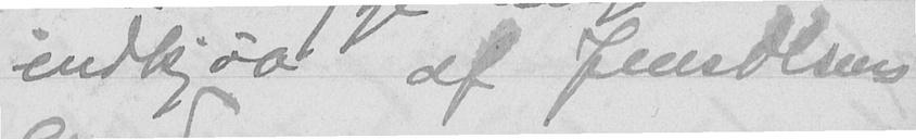indkjøb af Jens Olsens
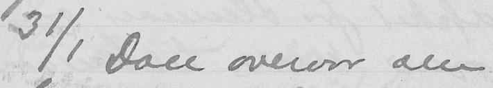31/1 Don overvar om
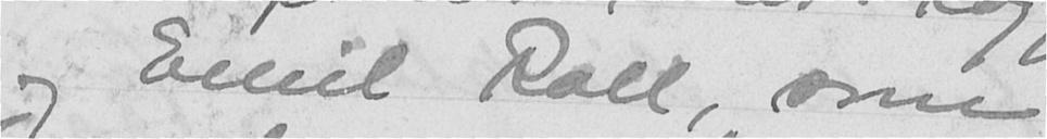og Emil Roll, som
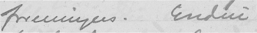foreningens. Endnu
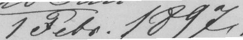1 Febr. 1897.
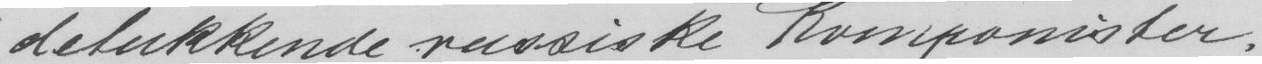delukkende russiske Komponister.
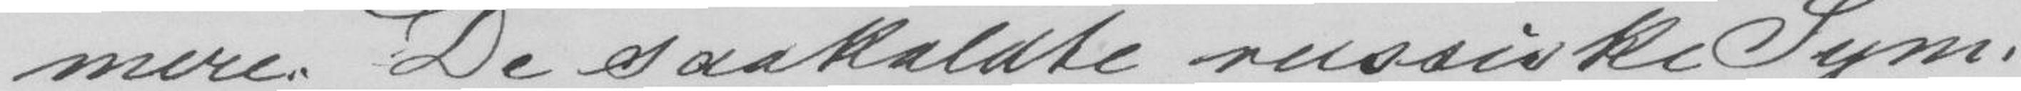mere.De saakaldte russiske Sym-
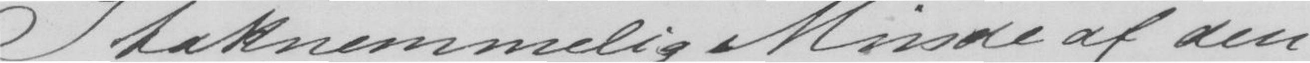I taknemmelig Minde af den
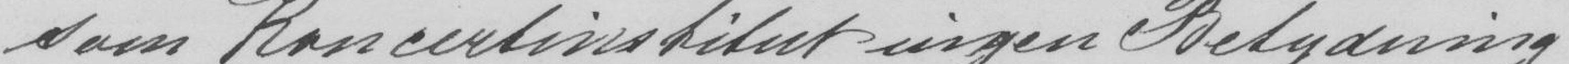som Koncertinstitut ingen Betydning
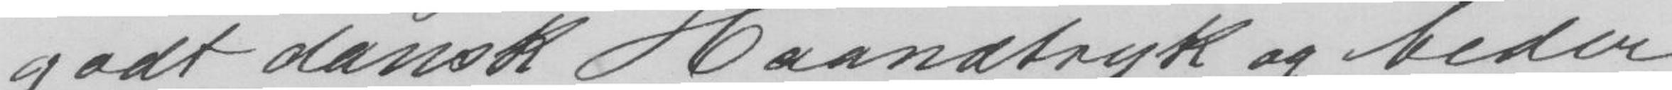godt dansk Haandtryk og beder
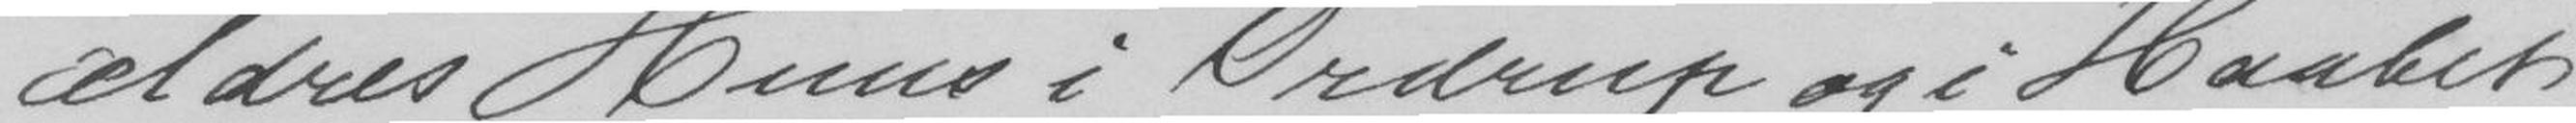ældres Huus i Orderup og i Haabet
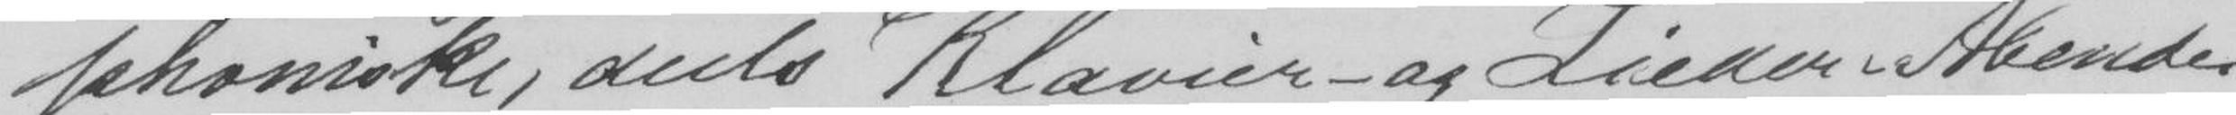phoniske, deels Klavier- og Lieder-Abende.
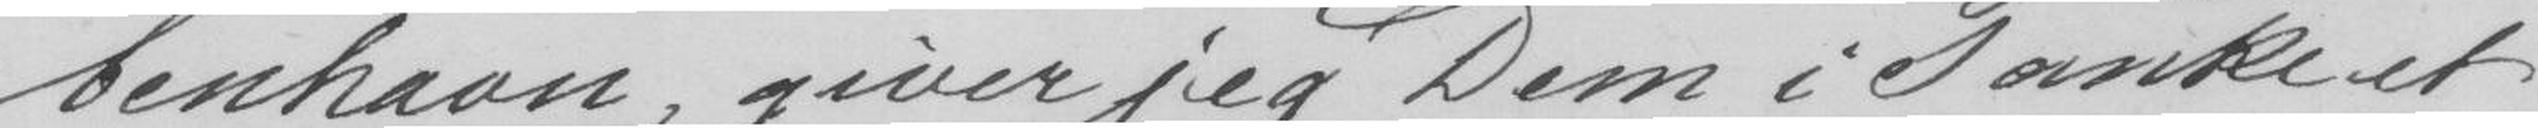benhavn, giver jeg Dem i Tanke et
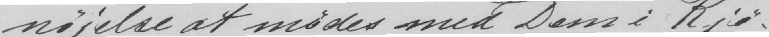nøjelse at mødes med Dem i Kjø-
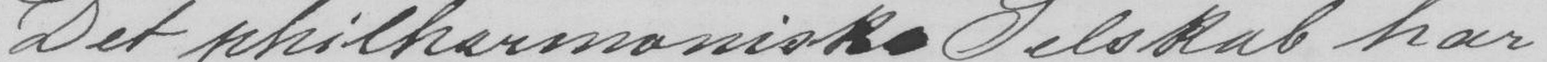Det philharmoniske Selskab har
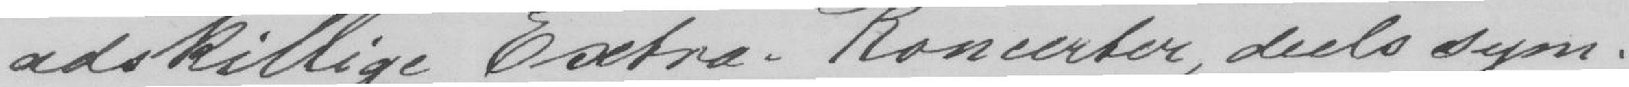adskillige Extra-Koncerter, deels sym-
